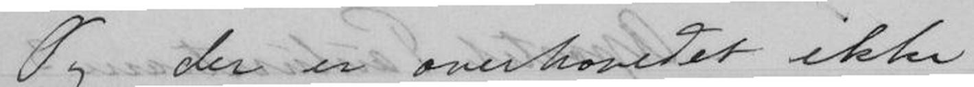Og der er overhovedet ikke
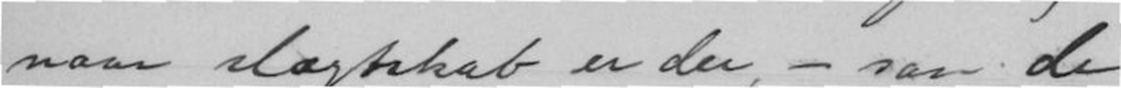naar slægtskab er der, - saa de
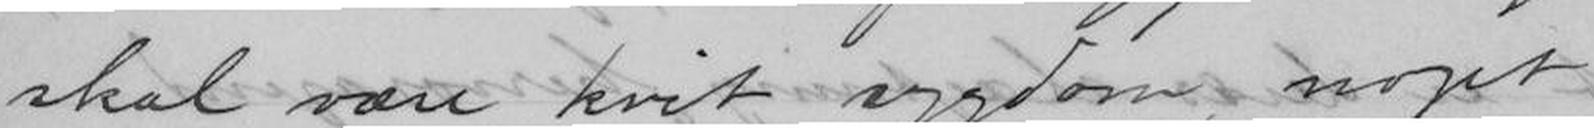skal være kvit sygdom, noget
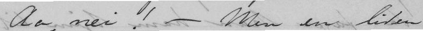- Aa, nei! - Men en liden
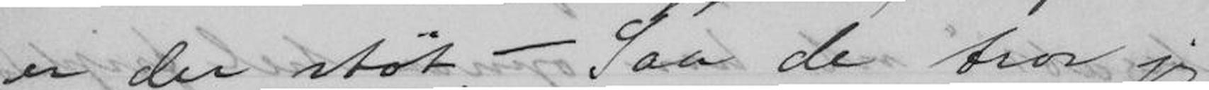er der støt. - Saa de tror jeg
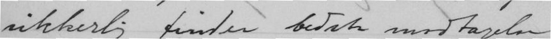sikkerlig finder bedst modtagelse
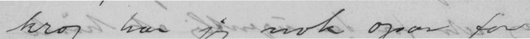krog har jeg nok ogsaa for
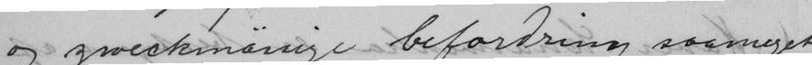og zweckmässige befordring saameget
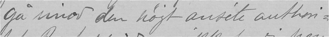gå imod den højt anséte autori-
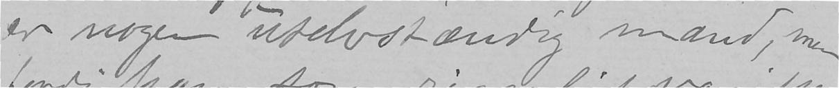er nogen uselvstændig mand, men
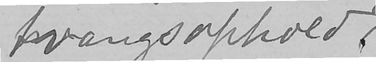tvangsophold
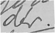der.
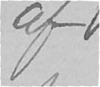af
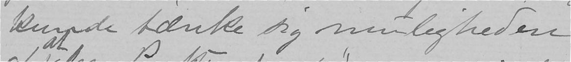kunde tænke sig muligheden
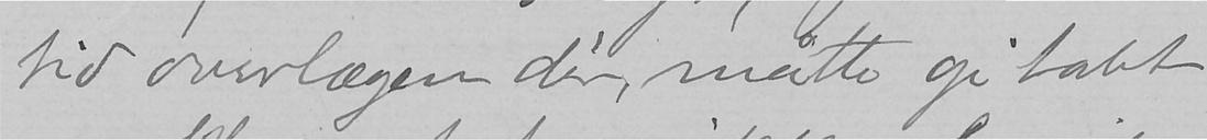tid overlægen der, måtte gi tabt
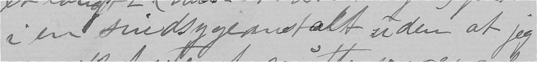i en sindsygeanstalt uden at jeg
